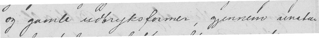og gamle udtryksformer, gjennem unatur
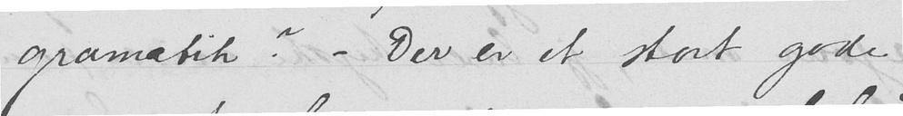gramatik? - Der er et stort gode
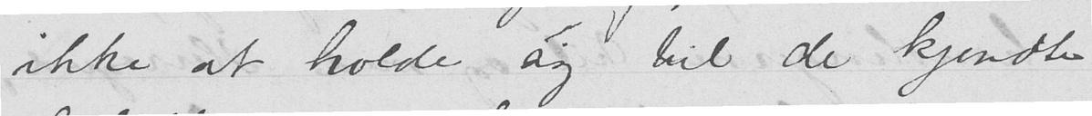ikke at holde sig til de kjendte
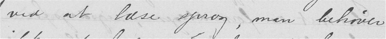ved at læse sprog, man behøver
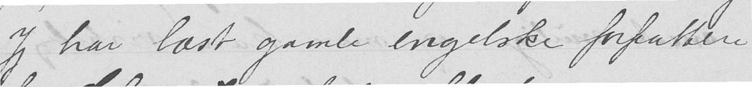Jeg har lest gamle engelske forfattere
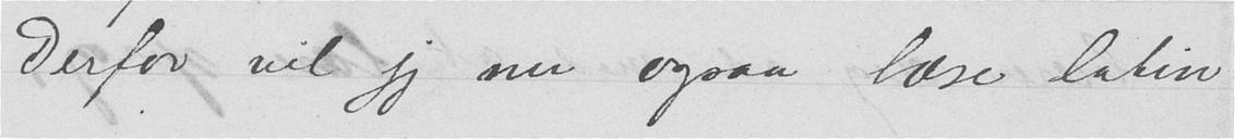Derfor vil jeg nu ogsaa læse latin
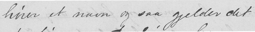hører et navn og saa gjelder det
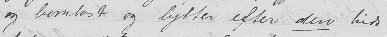og bombast og lytter efter den tids
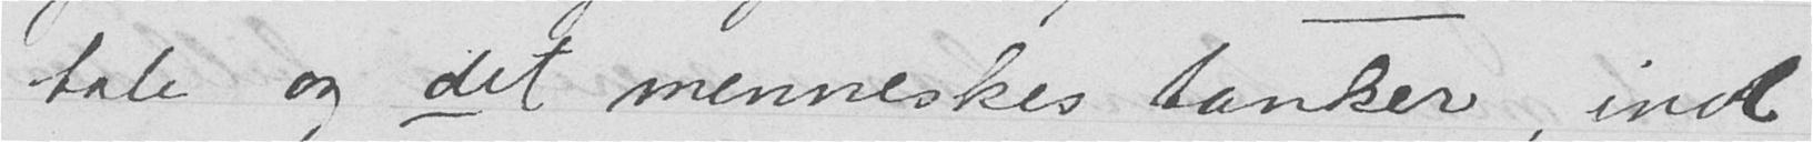tale og det menneskes tanker, ind
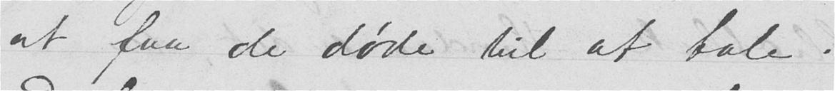at faa de døde til at tale.
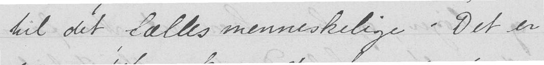til det fælles menneskelige. Det er
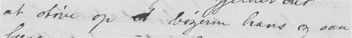at støve op  bøgerne hans og saa
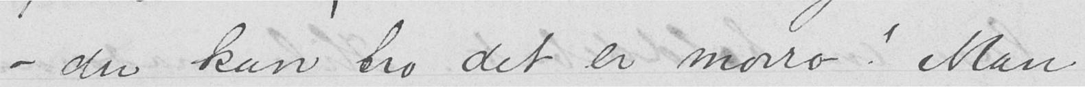- du kan tro det er morro! Man
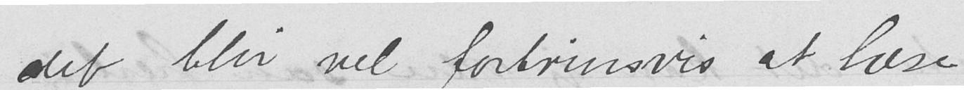det blir vel fortrinsvis at læse
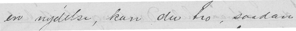en nydelse, kan du tro, saadan
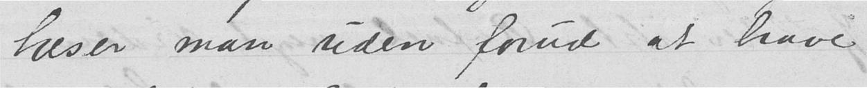læser man uden forud at have
